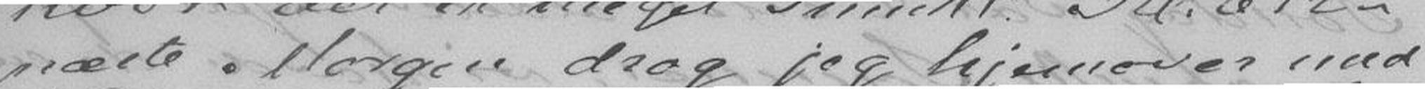næste Morgen drog jeg hjemover med
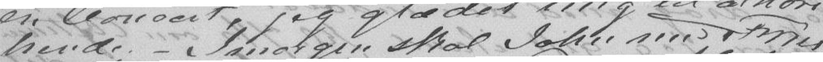hende. - Imorgen skal John med Fries
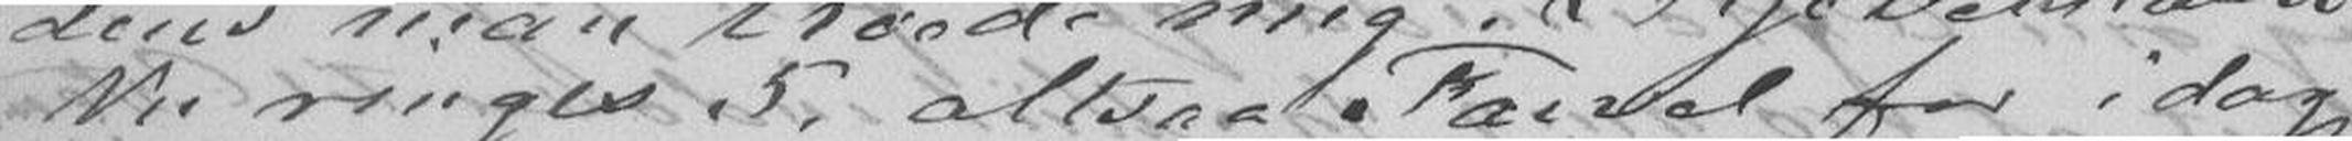Nu ringes 5, altsaa Farvel for idag
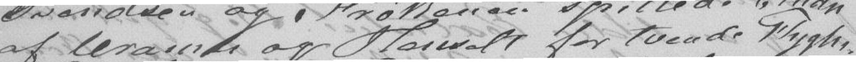af Cramer og Henselt for tvende Flygler.
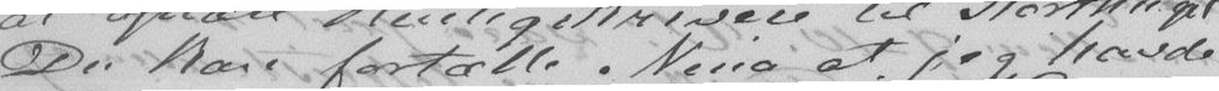Du kan fortælle Nina at jeg havde
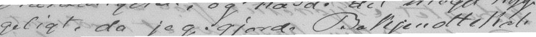geligt, da jeg gjorde Bekjendtskab
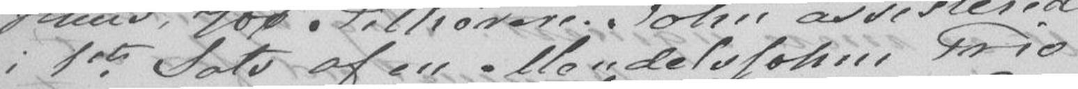i 1ste Sats af en Mendelssohn Trio
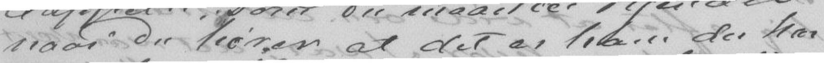naar Du hører at det er ham der har
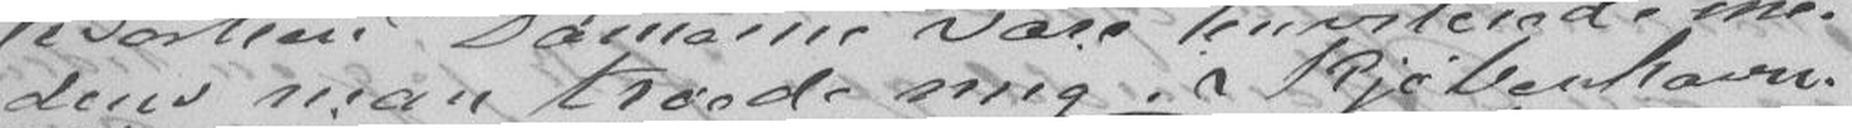dens man troede mig. Kjøbenhavn.
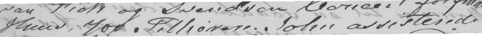Huus, 700 Tilhørere. John assisterede
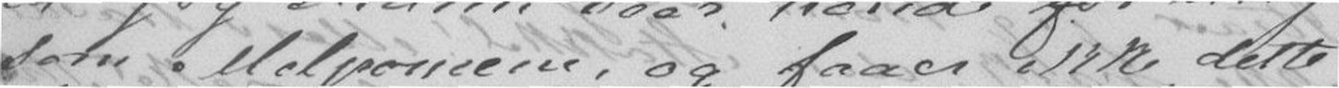som Melpoucene, og faaer ikke dette
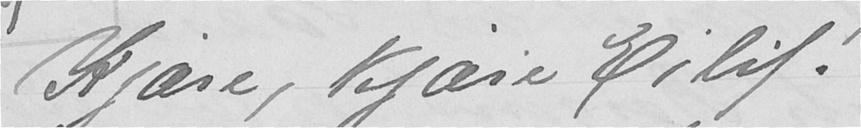Kjære, Kjære Eilif!
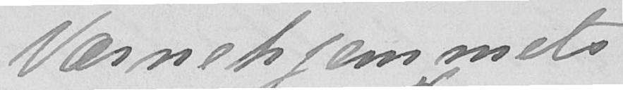"Værnehjemmets
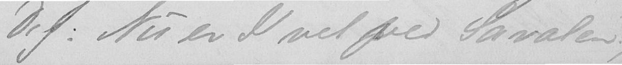Dig. Nu er I vel ved Savalen,
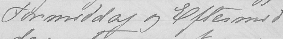Formiddag og Eftermid-
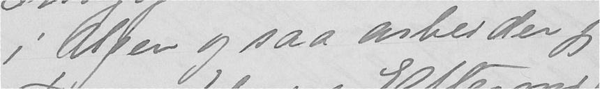i Ugen og saa arbeider jeg
